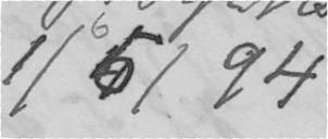1/5/94
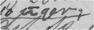i ti uger;
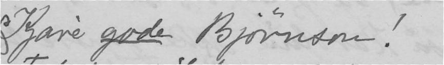Kjære gode Bjørnson!
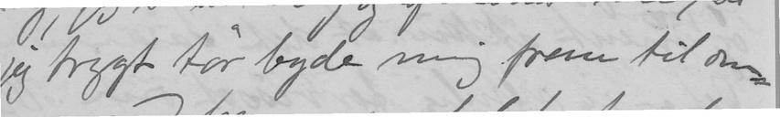jeg trygt får byde mig frem til om-
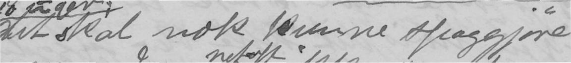det skal nok kunne spaggjøre
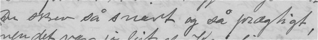Du skrev så snart og så prægligt,
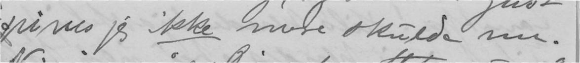pines jeg ikke mere skulde nu.
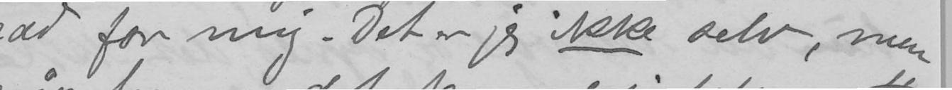ræd for mig. Det er jeg ikke selv, men
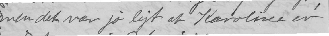men det var jo lejt at Karoline er
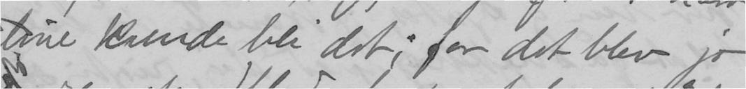line kunde bli det; for det blev jo
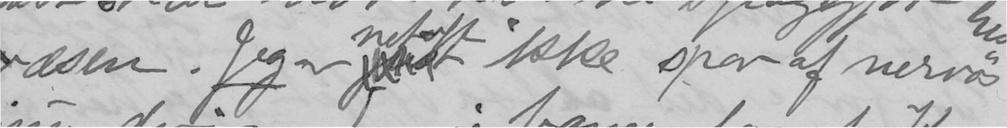væsen. Jeg er netop ikke spor af nervøs
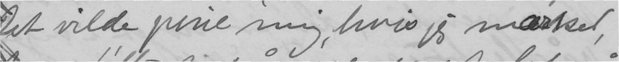Det vilde pine mig, hvis jeg mærked,
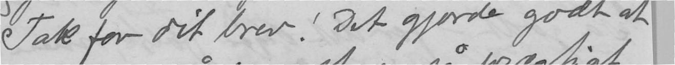Tak for dit brev! Det gjorde godt at
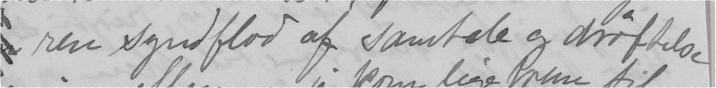ren syndflod af samtale og drøftelse
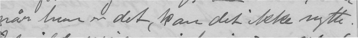når hun er det, kan det ikke nytte.
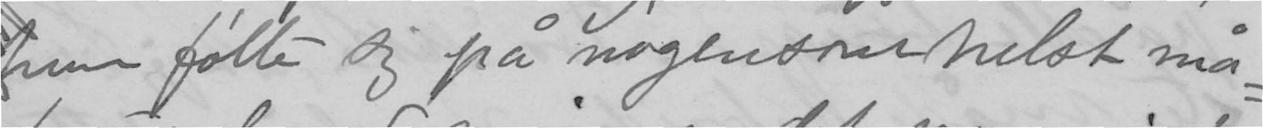hun følte sig på nogensomhelst må-
